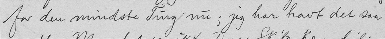for den mindste Ting nu; jeg har havt det saa
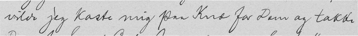vilde jeg kaste mig paa Knæ for Dem og takke
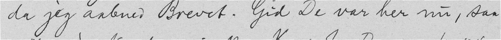da jeg aabned Brevet. Gid De var her nu, saa
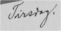Tirsdag.
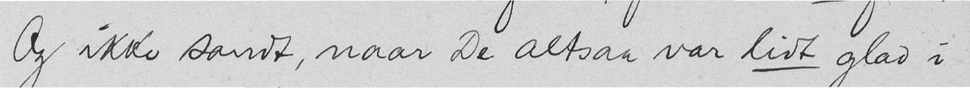Og ikke sandt, naar De altsaa var lidt glad i
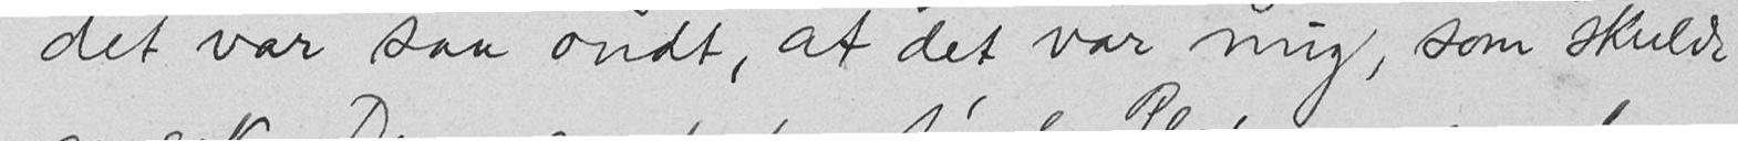det var saa ondt, at det var mig, som skulde
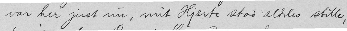var her just nu, mit Hjærte stod aldeles stille,
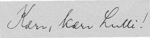Kære, kære Lulli!
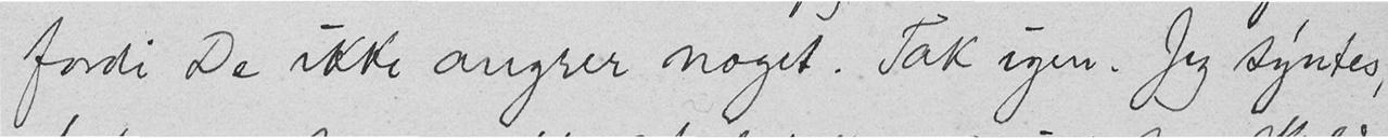fordi De ikke angrer noget. Tak igen. Jeg syntes,
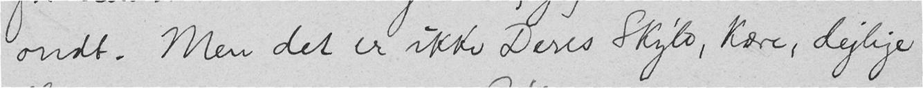ondt. Men det er ikke Deres Skyld, kære, dejlige
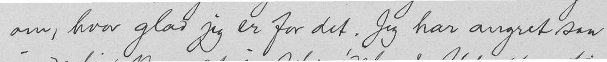om, hvor glad jeg er for det. Jeg har angret saa
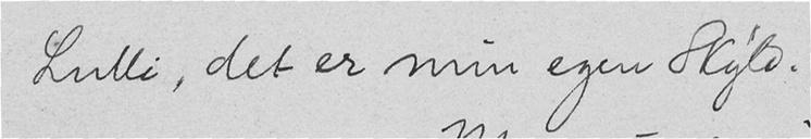Lulli, det er min egen Skyld.
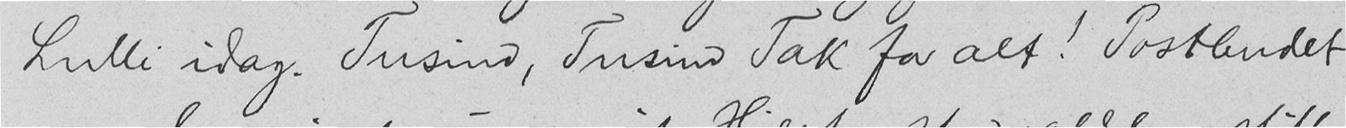Lulli idag. Tusind, Tusind Tak for alt! Postbudet
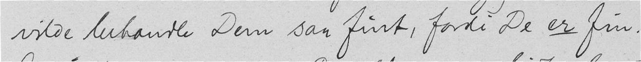vilde behandle Dem saa fint, fordi De er fin.
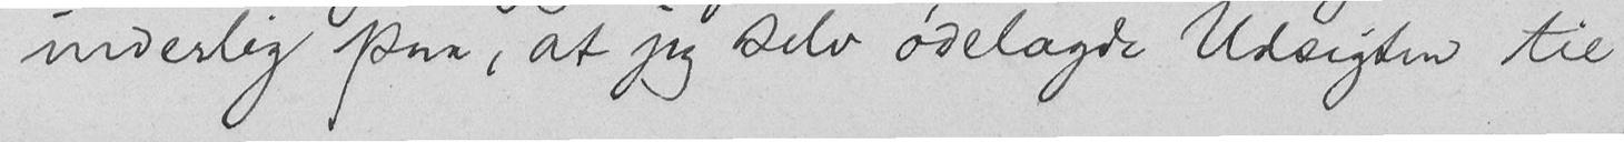inderlig paa, at jeg selv ødelagde Udsigten til
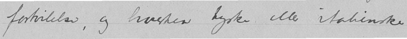fortvilelse, og hverken tyske eller italienske
